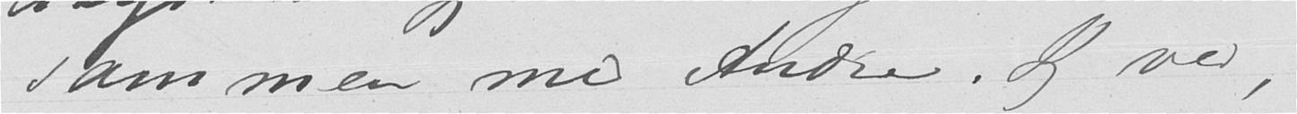sammen med Andre. Jeg ved,
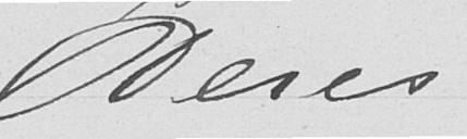Deres
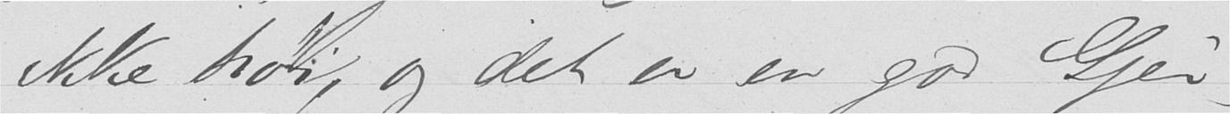ikke høi, og det er en god Gjer-
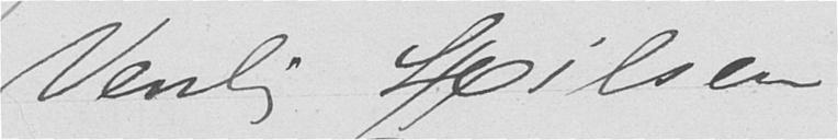Venlig Hilsen
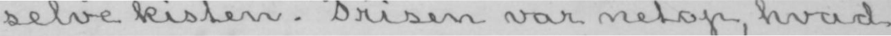selve kisten. Prisen var netop, hvad
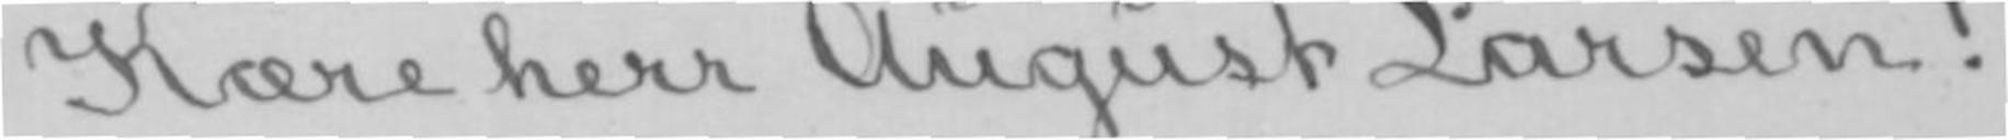Kære herr August Larsen!
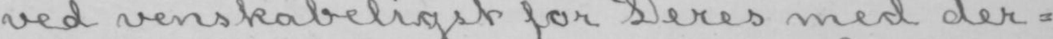ved venskabeligst for Deres med der-
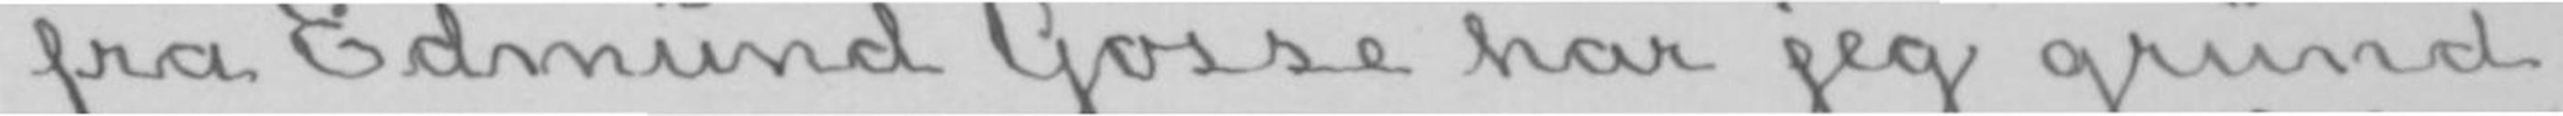fra Edmund Gosse har jeg grund
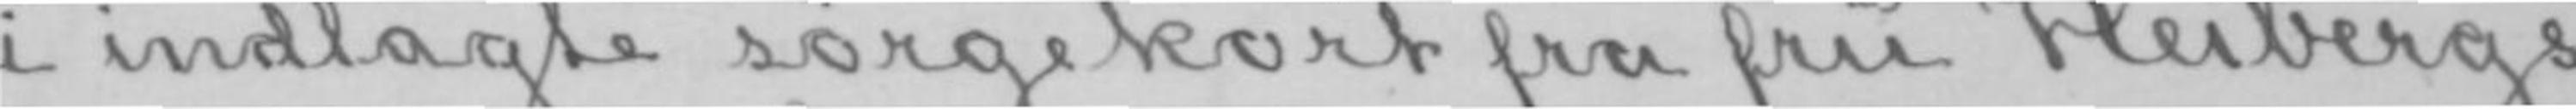i indlagte sørgekort fra fru Heibergs
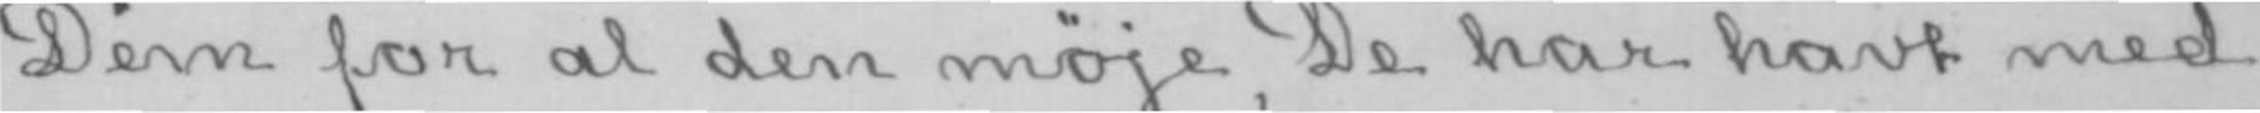Dem for al den møje, De har havt med
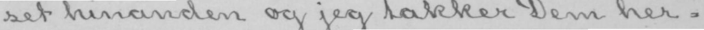set hinanden og jeg takker Dem her-
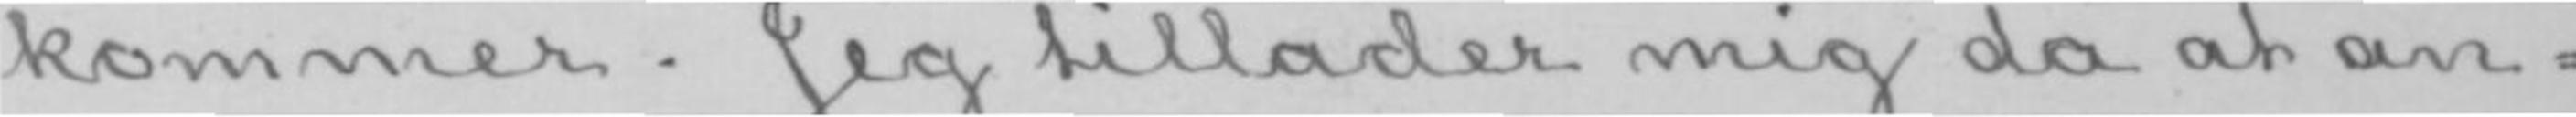kommer. Jeg tillader mig da at an-
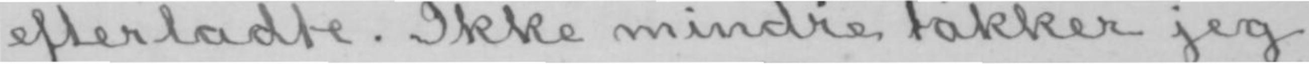efterladte. Ikke mindre takker jeg
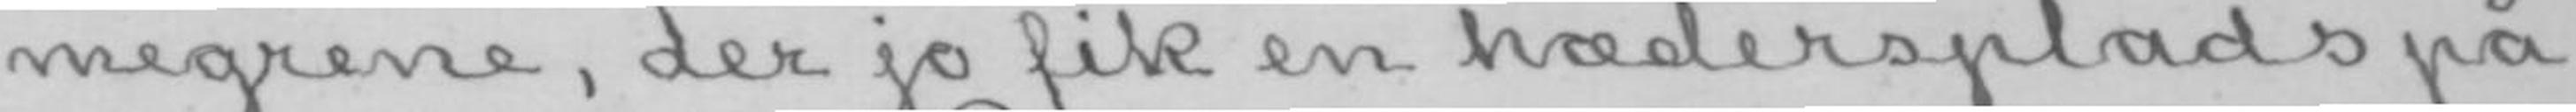megrene, der jo fik en hædersplads på
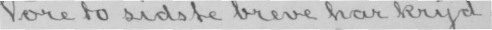Vore to sidste breve har kryd-
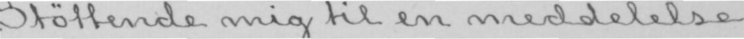Støttende mig til en meddelelse
